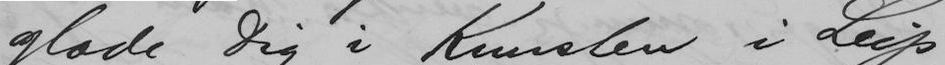glæde dig i Kunsten i Leip-
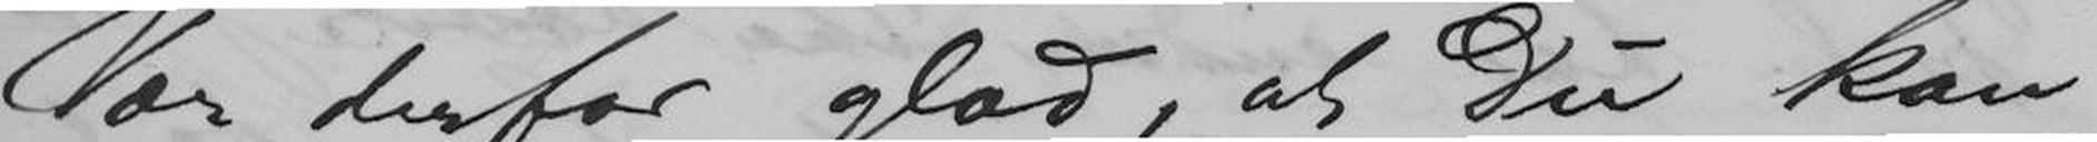Vær derfor glad, at Du kan
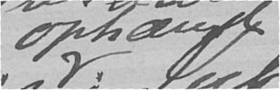ophængt
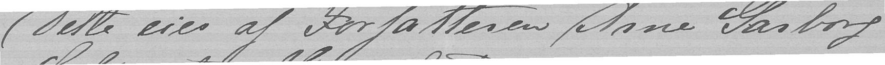Dette eies af Forfatteren Arne Garborg
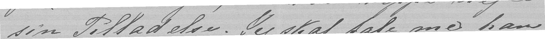sin Tilladelse. Jeg skal tale med ham
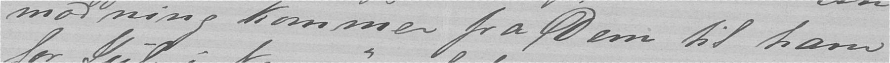modning kommer fra Dem til ham
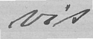vis
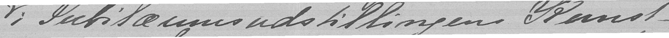i Jubilæumsudstillingens Kunst-
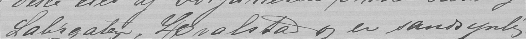Labraaten, Hvalstad og er sandsynlig-
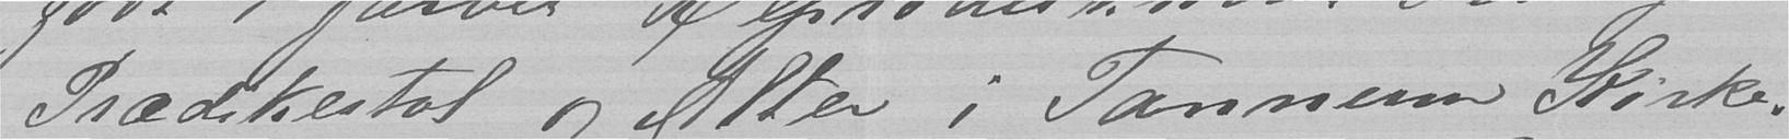Prædikestol og Alter i Tannum Kirke.
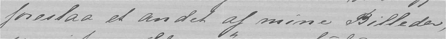foreslaa et andet af mine Billeder,
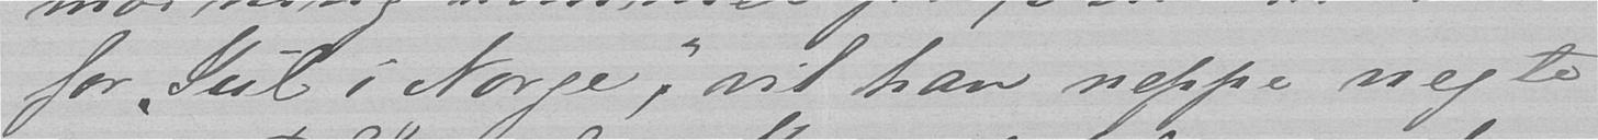for "Jul i Norge", vil han neppe negte
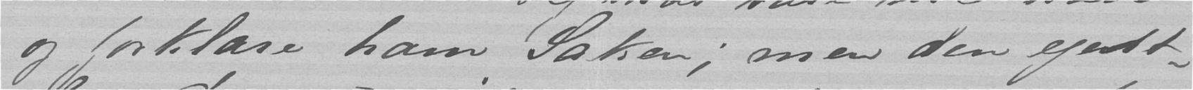og forklare ham Saken; men den egent-
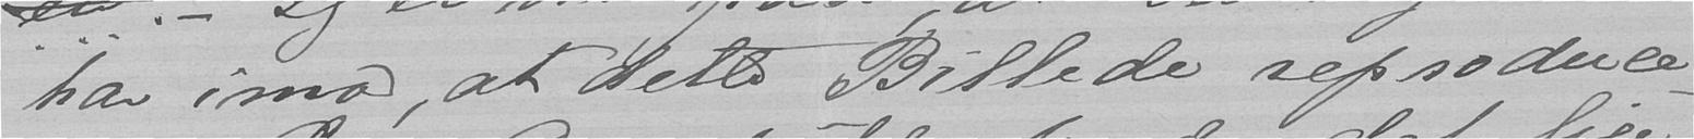har imod, at dette Billede reproduce-
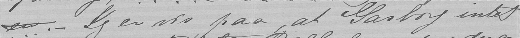en. - Jeg er vis paa at Garborg intet
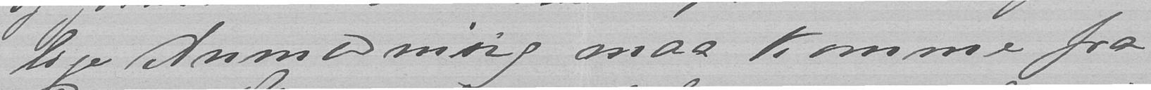lige Anmodning maa komme fra
